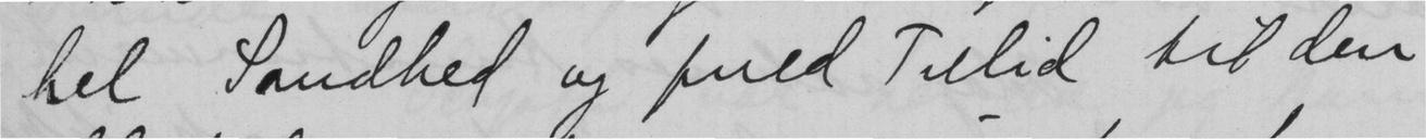hel Sandhed og fuld Tilid til den
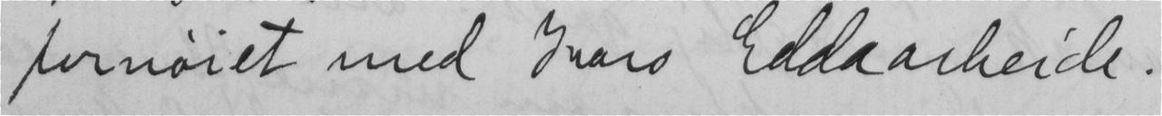fornøiet med Ivars Eddaarbeide.
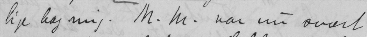lige bag mig. M. M. var nu svært
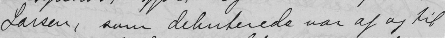Larsen, som debuterede var af og til
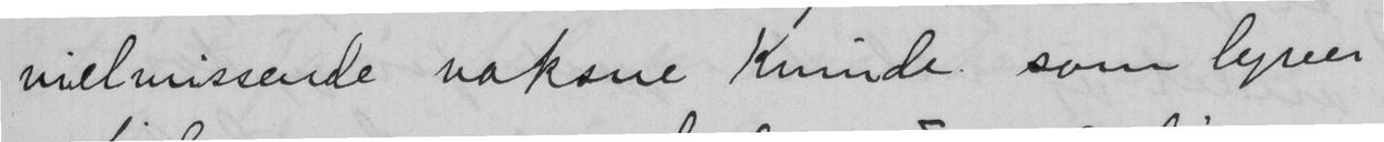vielmissende voksne Kvinde, som lyver
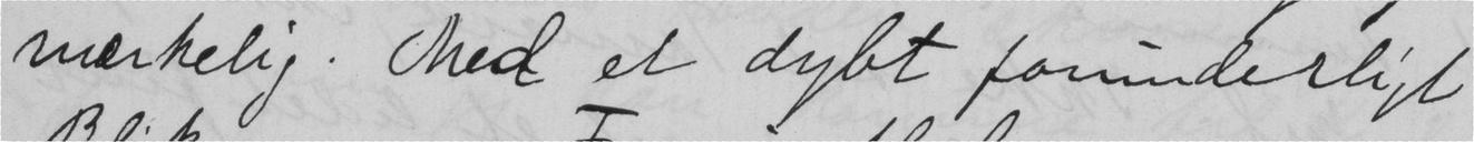mærkelig. Med et dybt forunderligt
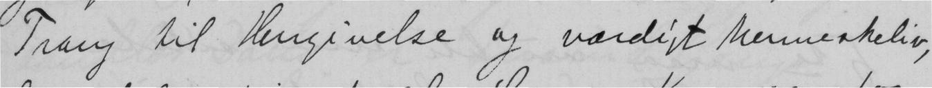Trang til Hengivelse og værdigt menneskeliv,
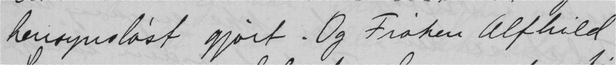hensynsløst gjort. Og Frøken Alfhild
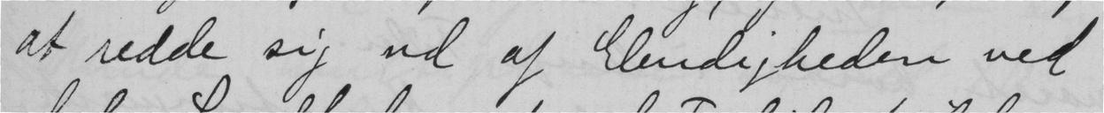at redde sig ud af Elendigheden ved
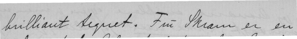brilliant tegnet. Fru Skram er en
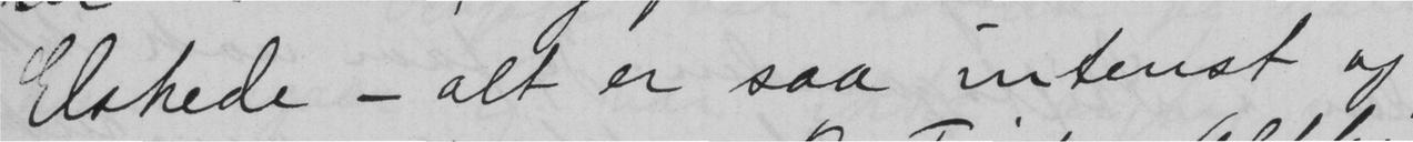Elskede - alt er saa intenst og
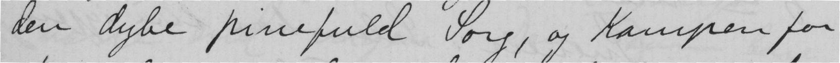den dybe pinefuld Sorg, og Kampen for
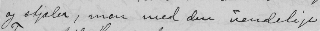og stjæler, men med den uendelige
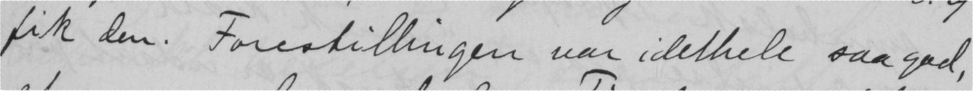fik den. Forestillingen var idethele saa god,
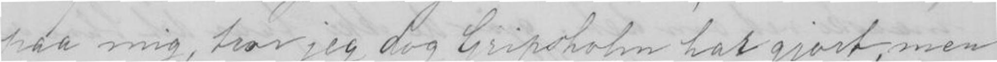paa mig, tror jeg dog Gripholm har gjort, men
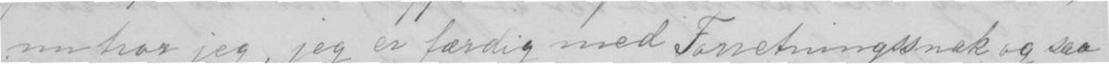no tror jeg, jeg er færdig med Forsetningssnak og saa
